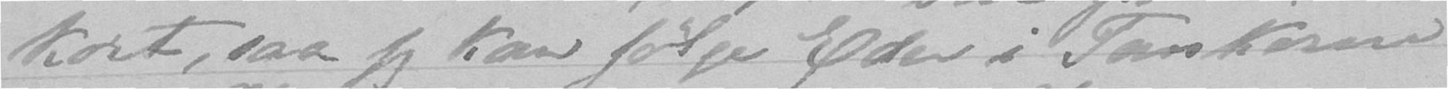kort, saa jeg kan følge Eder i Tankerne
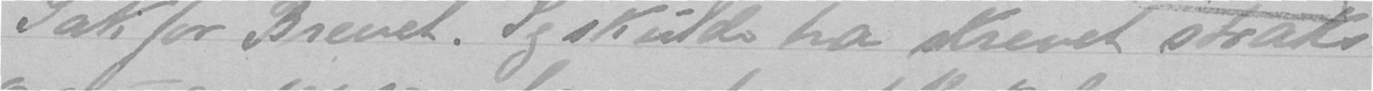Tak for Brevet. Jeg skulde ha skrevet straks
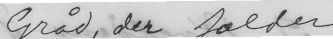Gråd, der falder,
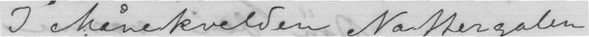I Månekvelden Nattergalen
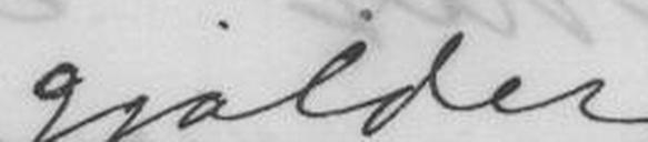gjalder,
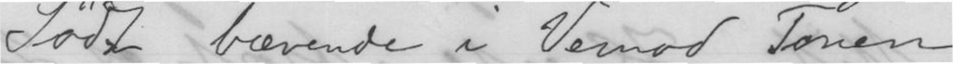Sødt bærende i Vemod Tonen
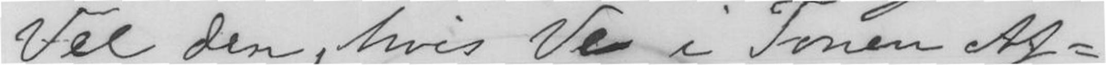Vel den, hvis Ve i Tonen af-
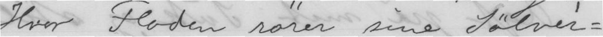Hvor Floden rører sine Sølver-
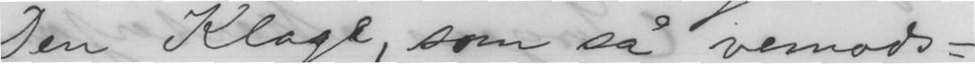Den Klage, som så vemods-
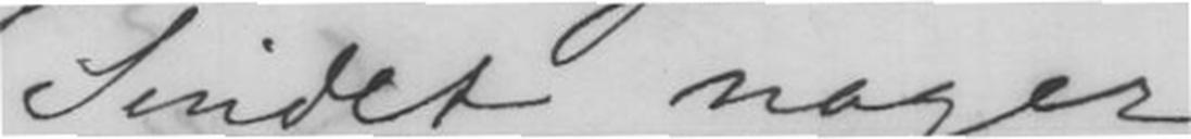Sindet nager,
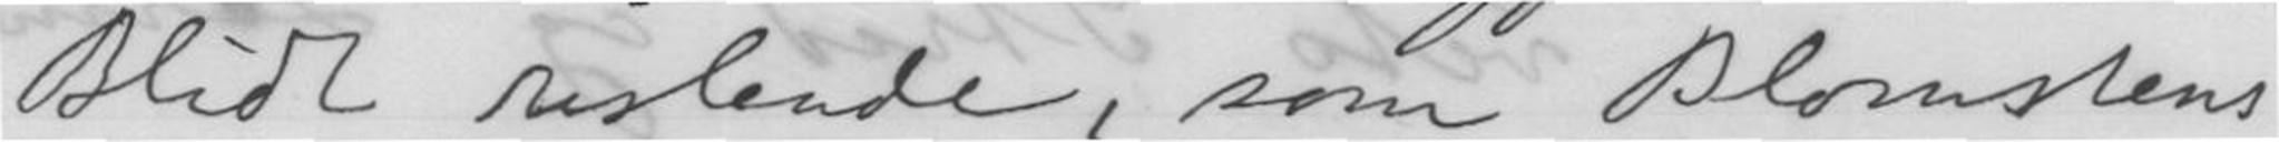Blidt rislende, som Blomstens
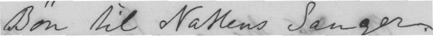Bøn til Nattens Sanger
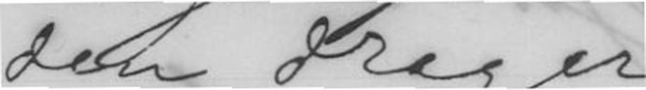den drager
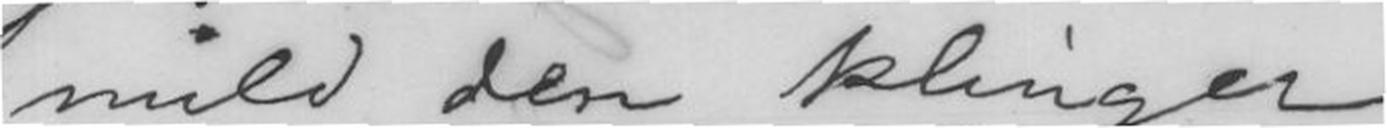mild den klinger.
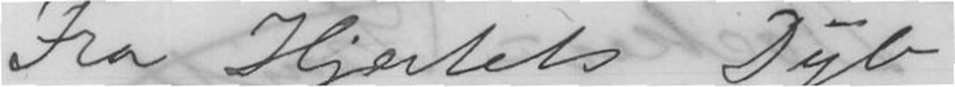Fra Hjertets Dyb
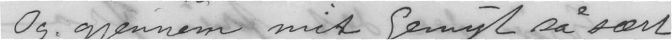Og gjennem mit Gemyt så sært
